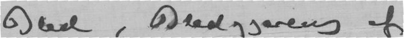Blod, Blodgjæreng af
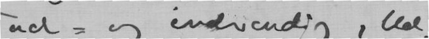ud= og indvændig, Ud-
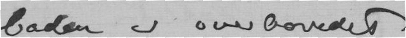baden er overhovedet
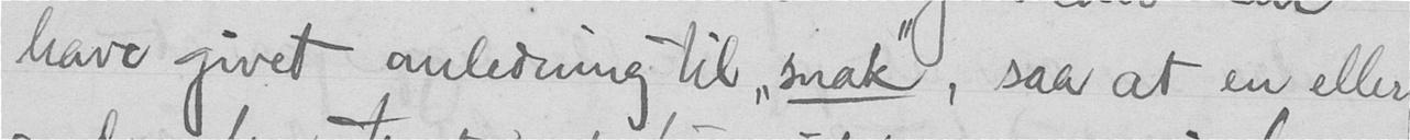have givet anledning til "snak", saa at en eller
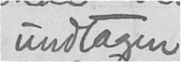undtagen
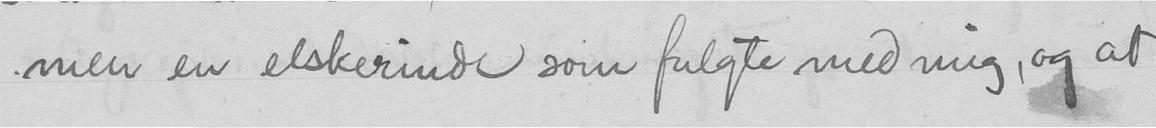men en elskerinde som fulgte med mig, og at
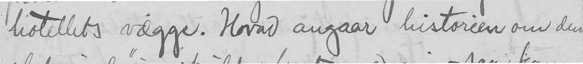hotellets vægge. Hvad angaar historien om den
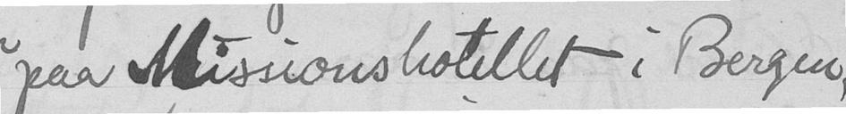paa Missionshotellet i Bergen,
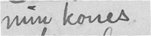min kones
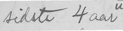sidste 4 aar
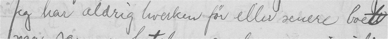Jeg har aldrig hverken før eller senere boet
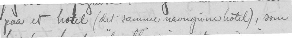paa et hotel (det samme navngivne hotel), som
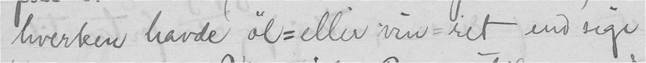hverken havde øl-eller vin-ret end sige
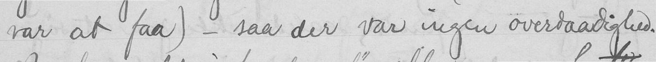var at faa), - saa der var ingen overdaadighed.
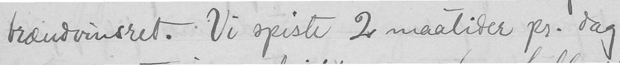trændvinsret. Vi spiste 2 maaltider pr. dag
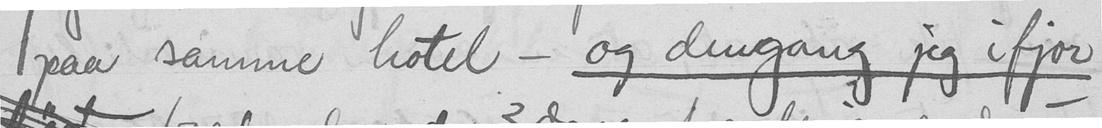paa samme hotel - og dengang jeg ifjor
